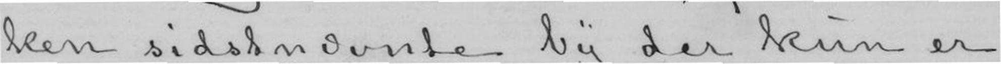ken sidstnævnte by der kun er
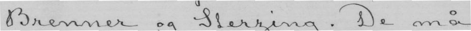Brenner og Sterzing. De må
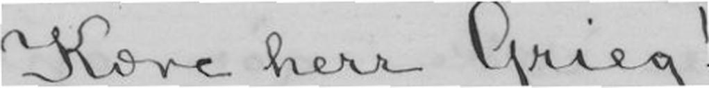Kære herr Grieg!
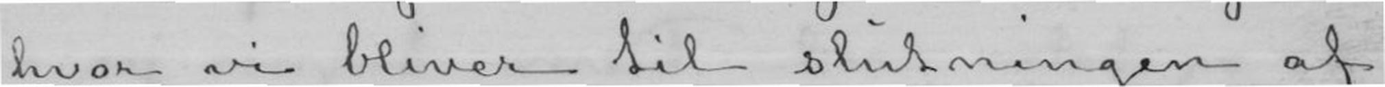hvor vi bliver til slutningen af
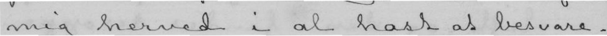mig herved i al hast at besvare.
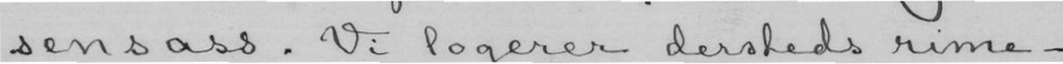sensass. Vi logerer dersteds rime-
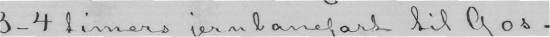3-4 timers jernbanefart til Gos-
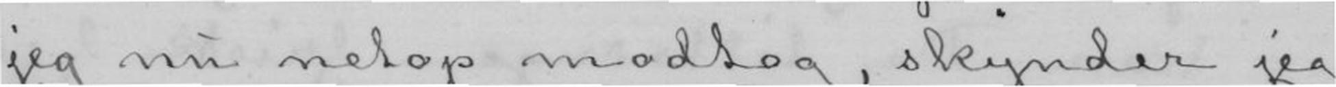jeg nu netop modtog, skynder jeg
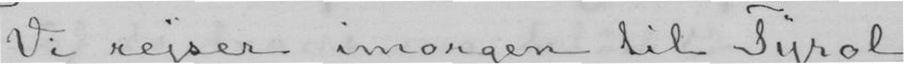Vi rejser imorgen til Tyrol,
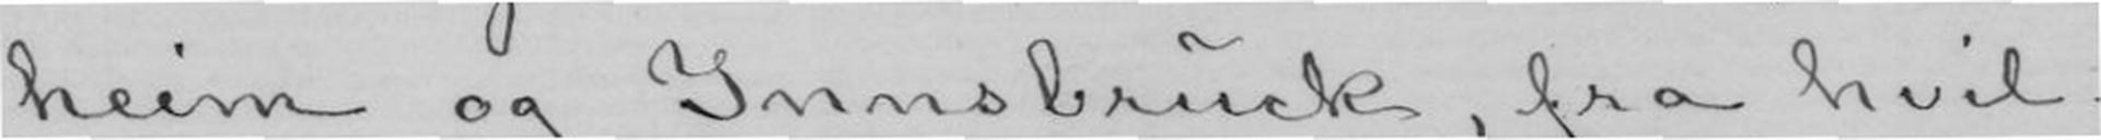heim og Innsbruck, fra hvil-
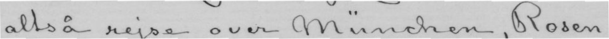altså rejse over Munchen, Rosen-
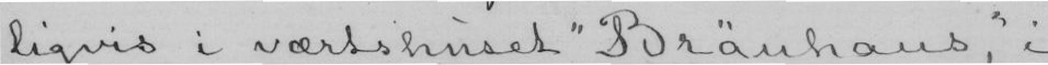ligvis i værtshuset "Bräuhaus", i
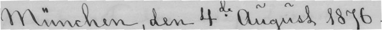Munchen, den 4de August 1876.
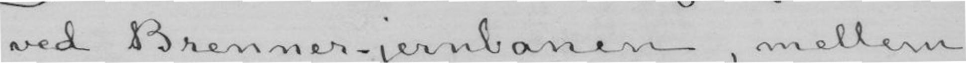ved Brenner-jernbanen, mellem
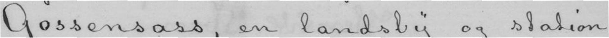Gossensass, en landsby og station
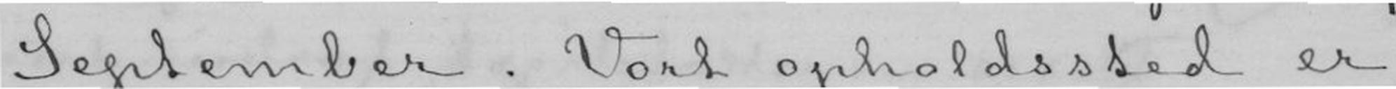September. Vort opholdsted er
